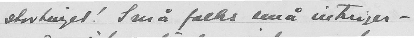stortinget! Små folks små intriger -
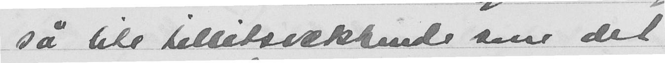så lite tilletsvækkende som det
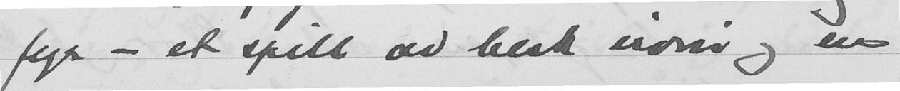fyr - et spill av besk ironi og en
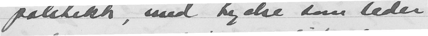politikk, med hegelse som leder
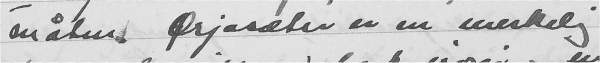måten. Ørjasæter er en merkelig
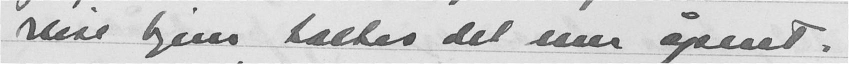reise hjem taltes det mer åpent.
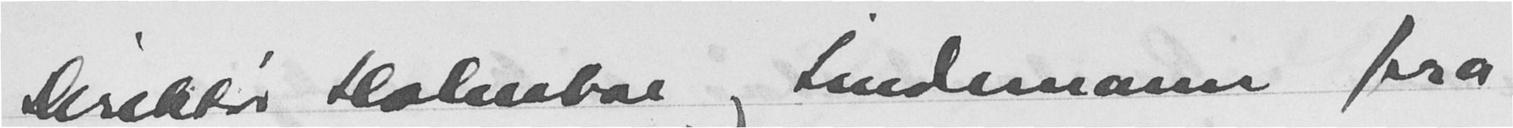Direktør Holmboe og Tindemann fra
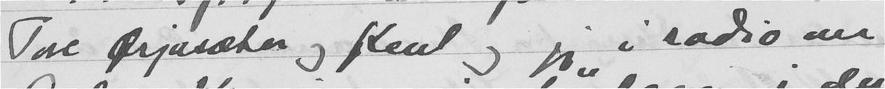Tore Ørjasæter og Kent og jeg i radio om
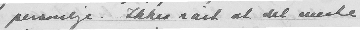personlige. Ikke rart at det meste
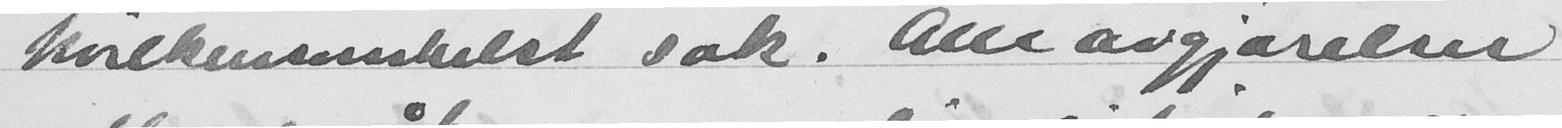hvilkensomshelst sak. Alle avgjørelser
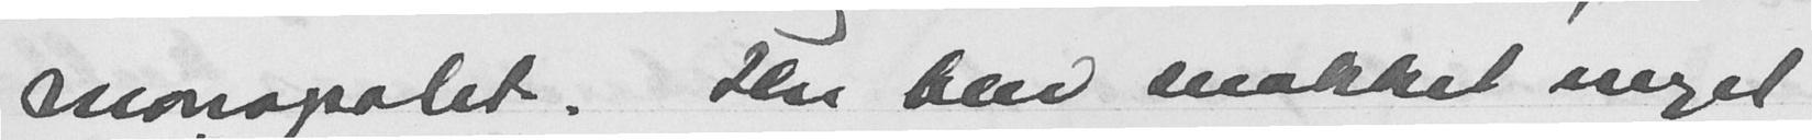monopolet. Her blir snakket meget
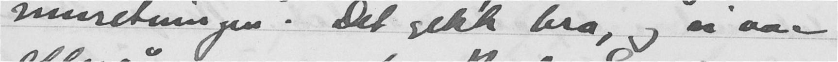innretningen. Det gikk bra, og vi var
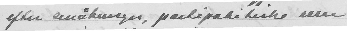efter småkneseger, partipolitiske eller
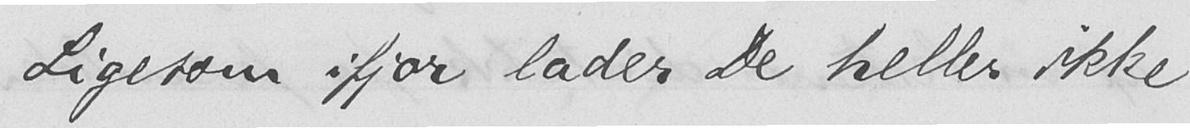Ligesom ifjor lader De heller ikke
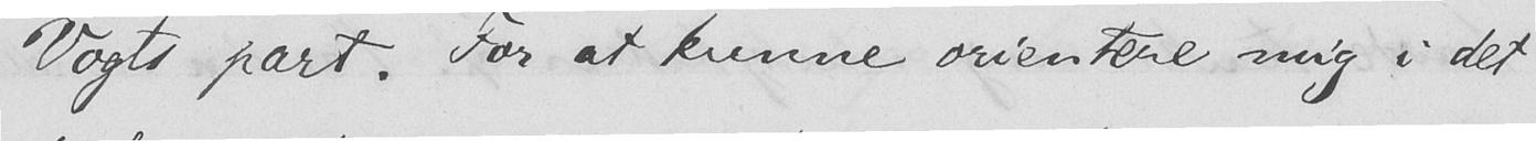Vogts part. For at kunne orientere mig i det
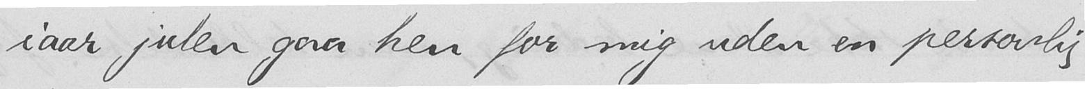iaar julen gaa hen for mig uden en personlig
